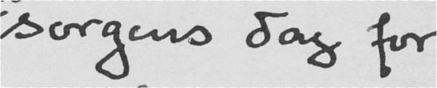sorgens dag for
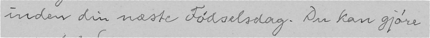inden din næste Fødselsdag. Du kan gjøre
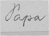Papa
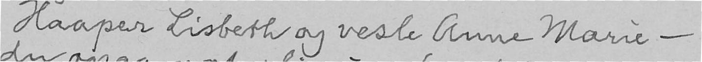Haaper Lisbeth og vesle Anne Marie -
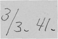3/3. 41.
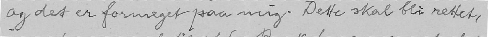og det er formeget paa mig. Dette skal bli rettet,
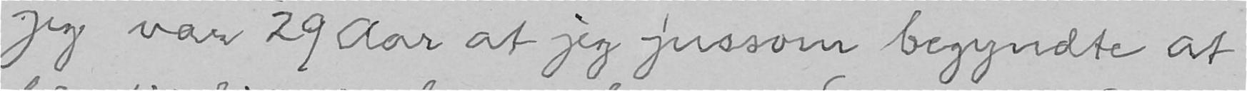jeg var 29 Aar at jeg jussom begyndte at
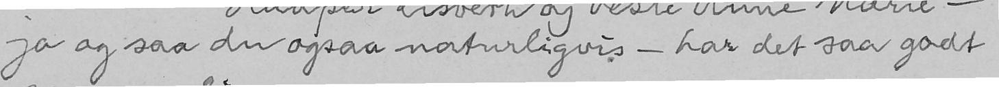ja og saa du ogsaa naturligvis - har det saa godt
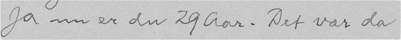Ja nu er du 29 Aar. Det var da
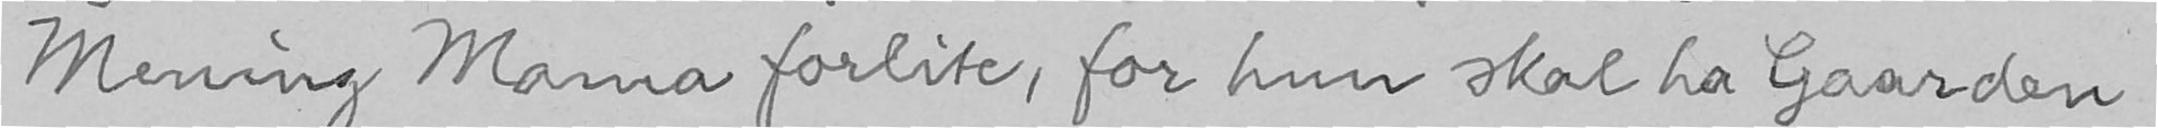Mening Mama forlite, for hun skal ha Gaarden
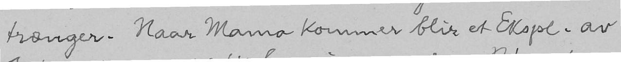trænger. Naar Mama kommer blir et Ekspl. av
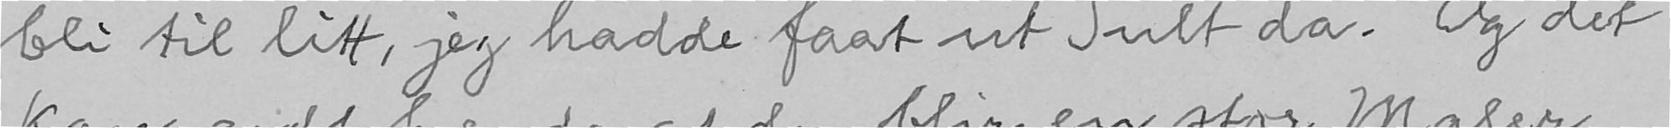bli til litt, jeg hadde faat ut Sult da. Og det
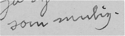som mulig.
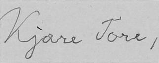Kjære Tore,
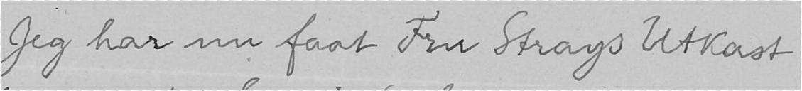Jeg har nu faat Fru Strays Utkast
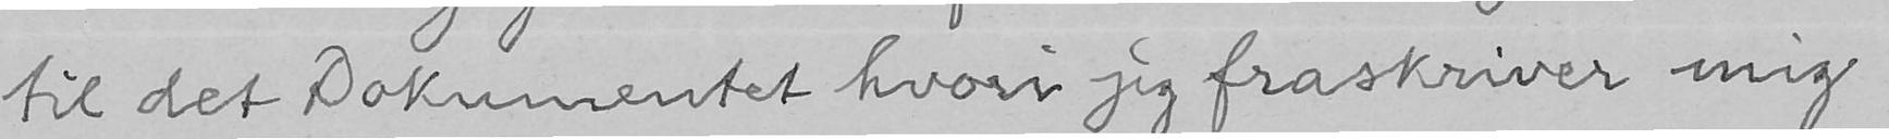til det Dokumentet hvori jeg fraskriver mig
